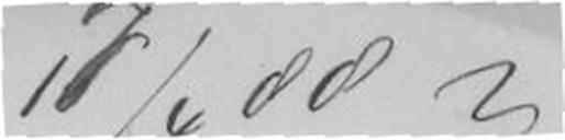17/4 88 ?
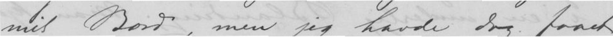mit Bord, men jeg havde dog faaet
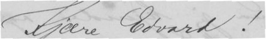Kjære Edvard!
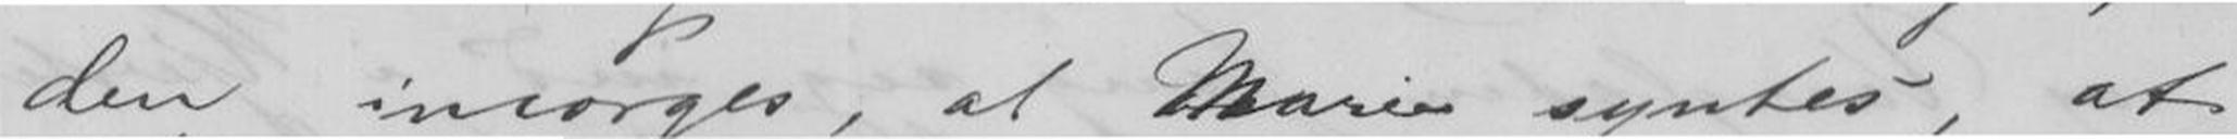den imorges, at Marie syntes, at
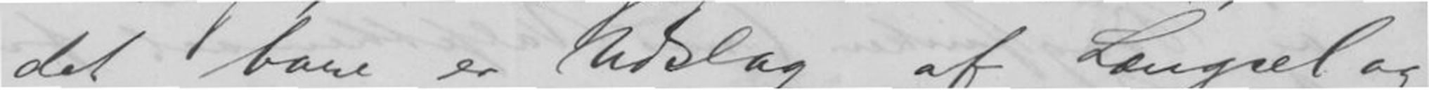det bare er Udslag af Længsel og
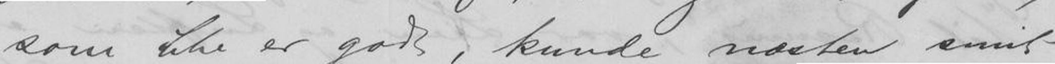som ikke er godt, kunde næsten smit-
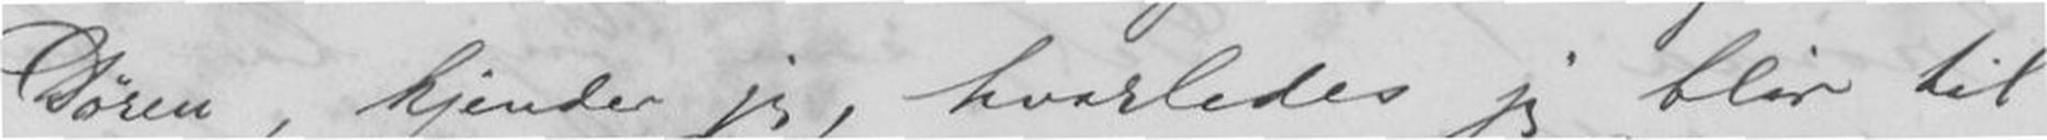Døren, kjender jeg, hvorledes jeg blir til
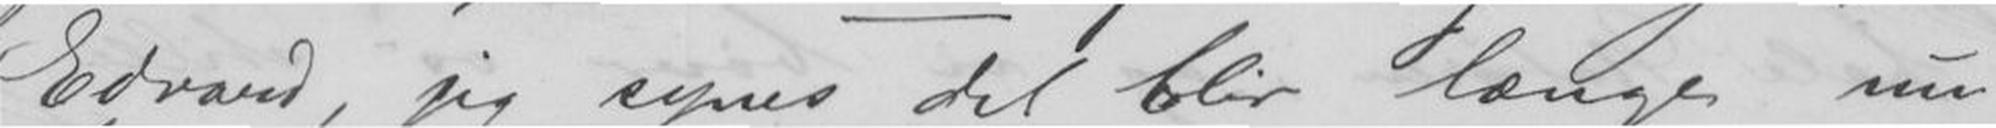Edvard, jeg synes det blir længe nu
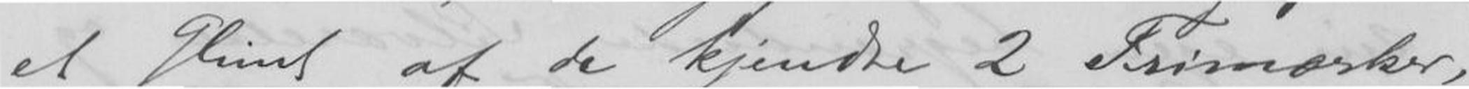et Glimt af de kjendte 2 Frimærker,
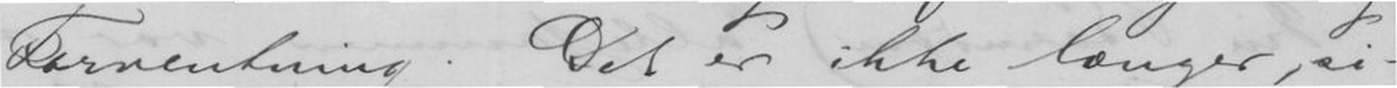Forventning. Det er ikke længer, si-
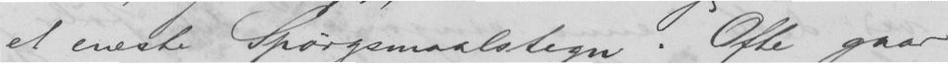et eneste Spørgsmaalstegn. Ofte gaar
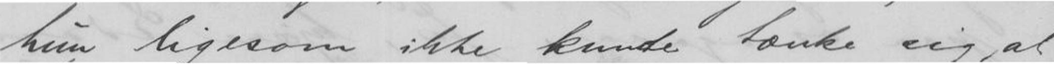hun ligesom ikke kunde tænke sig, at
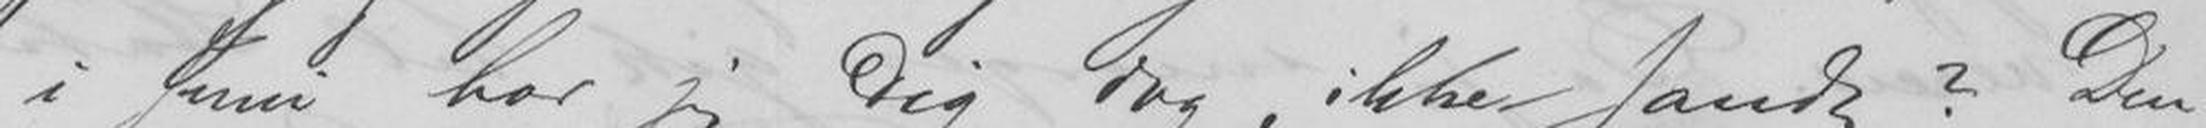i Juni har jeg Dig dog, ikke sandt? Den
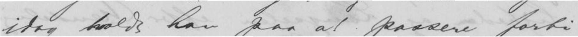idag holdt han paa at passere forbi
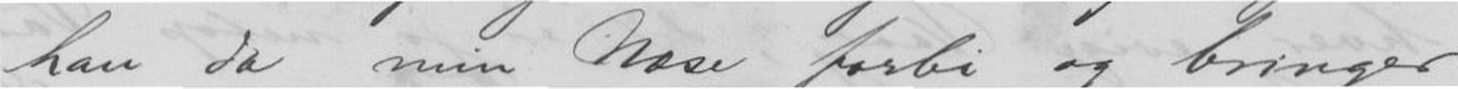han da min Næse forbi og bringer
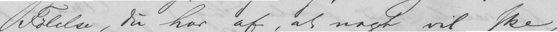Følelse, Du har af, at noget vil ske,
